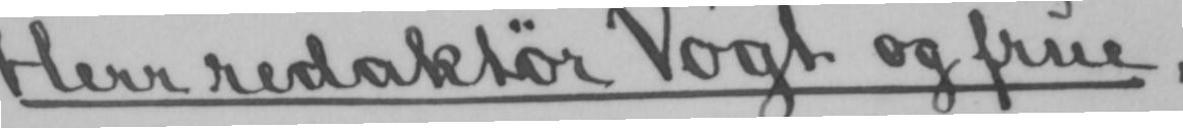Herr redaktør Vogt og frue,
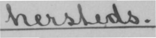hersteds.
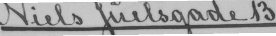Niels Juelsgade 13
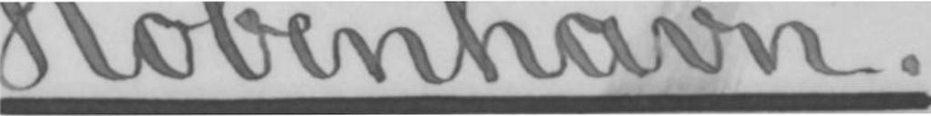København.
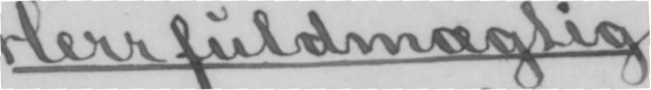Herr fuldmægtig
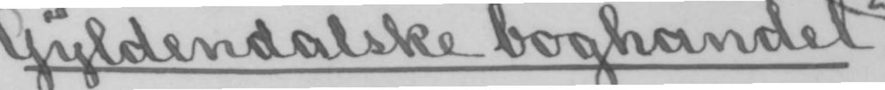Gyldendalske boghandel,
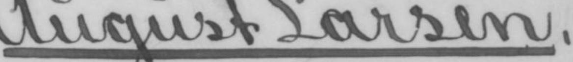August Larsen,
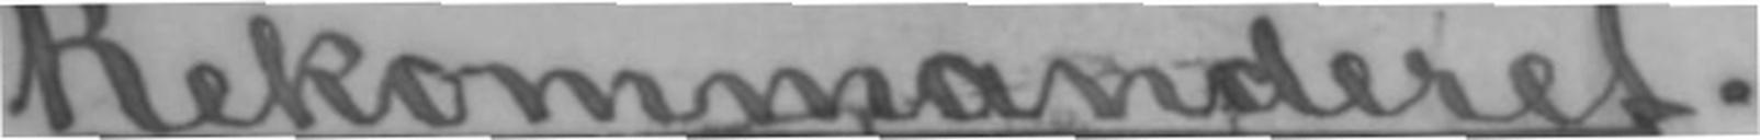Rekommanderet
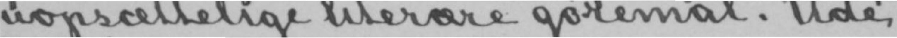uopsættelige literære gøremål. Ude
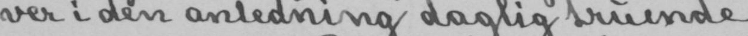ver i den anledning daglig truende
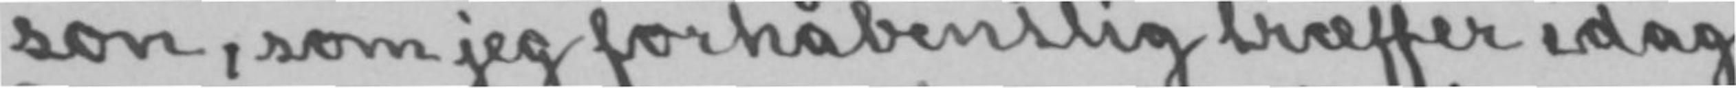son, som jeg forhåbentlig træffer idag.
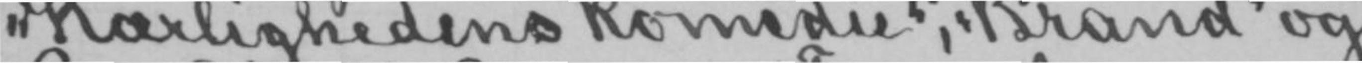«Kærlighedens komedie», «Brand» og
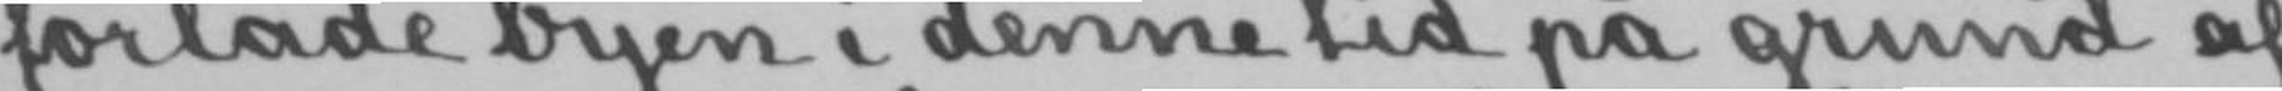forlade byen i denne tid på grund af
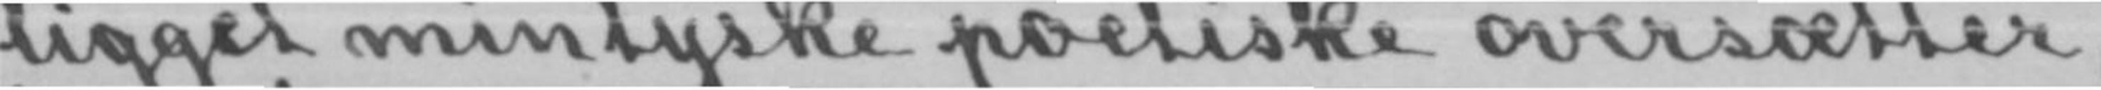ligget min tyske poetiske oversætter,
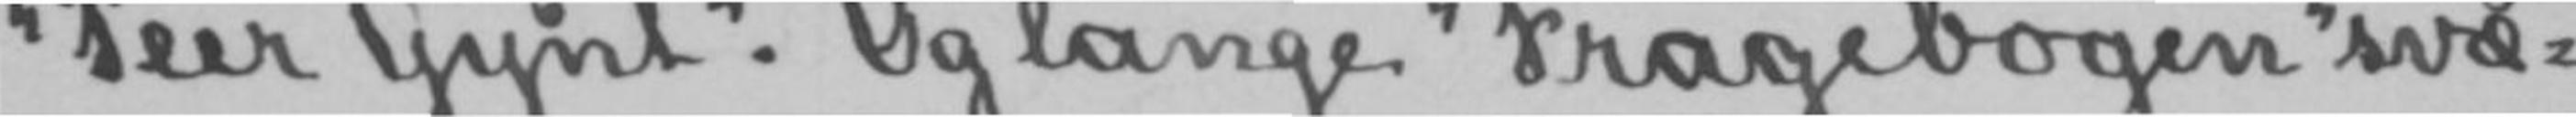«Peer Gynt». Og lange «Fragebogen» svæ-
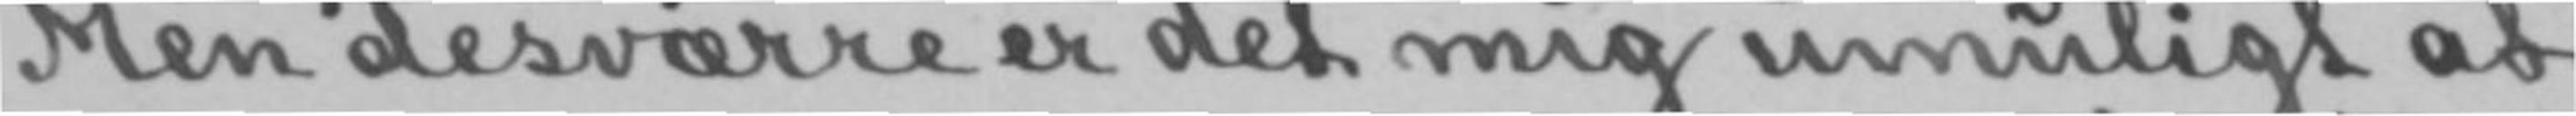Men desværre er det mig umuligt at
